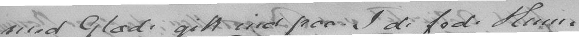med Glæde gik ind paa I de fede Hum-
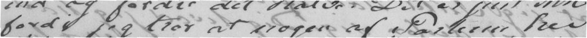fordi jeg tror at nogen af Partene her
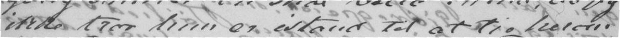ikke tror hun er istand til at tie herom
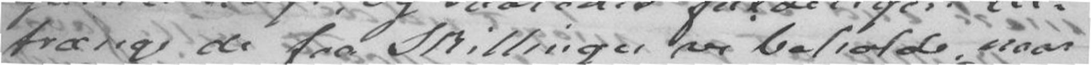trænge de faa Skilling vi beholde naar
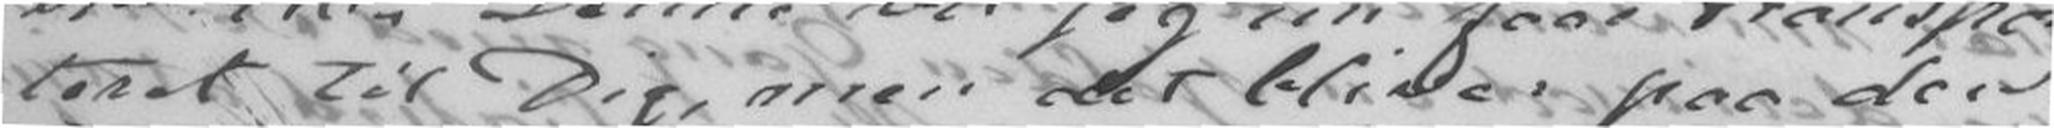teret til Dig, men det bliver paa den
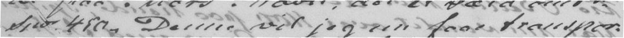Spd. 450. Denne vil jeg nu faae transpor-
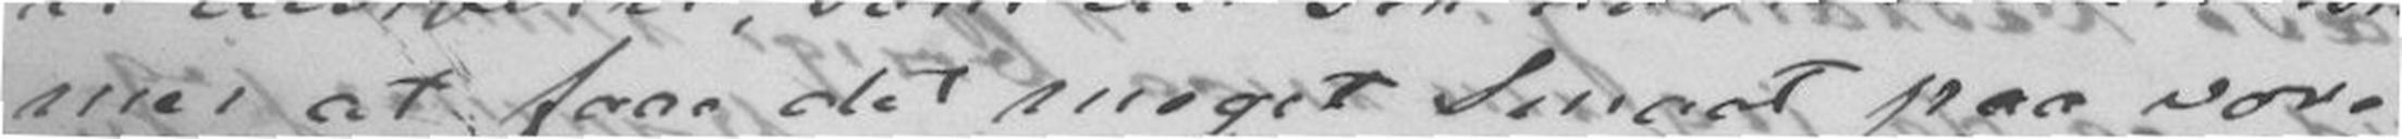mer at faae det meget Smaat paa vore
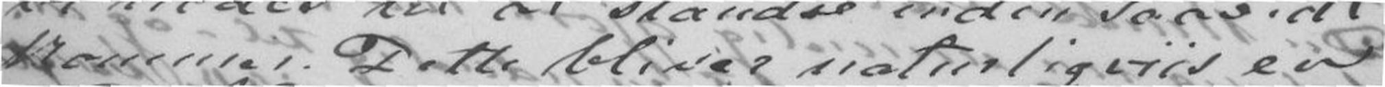kommer. Dette bliver naturligviis en
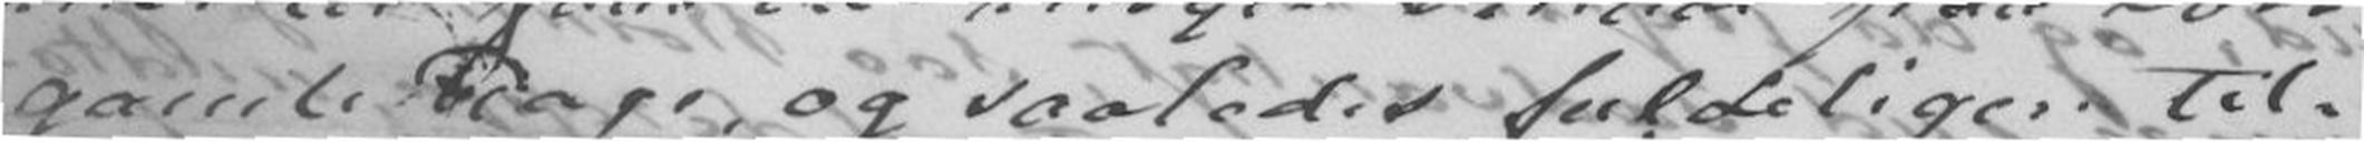gamle Dage, og saaledes fuldeligen til-
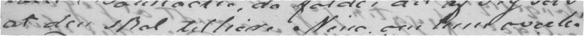at den skal tilhøre Nina om hun overle-
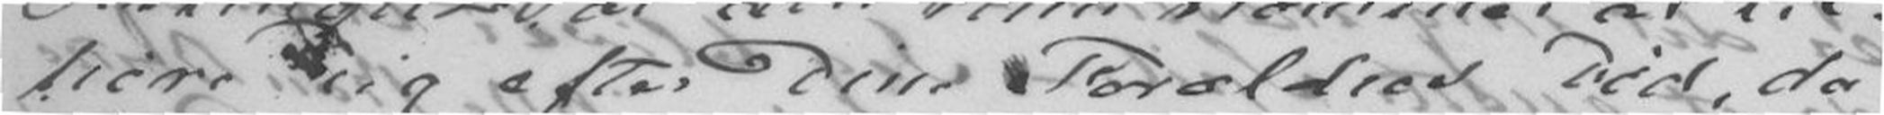høre Dig efter Dine Forældres Død, da
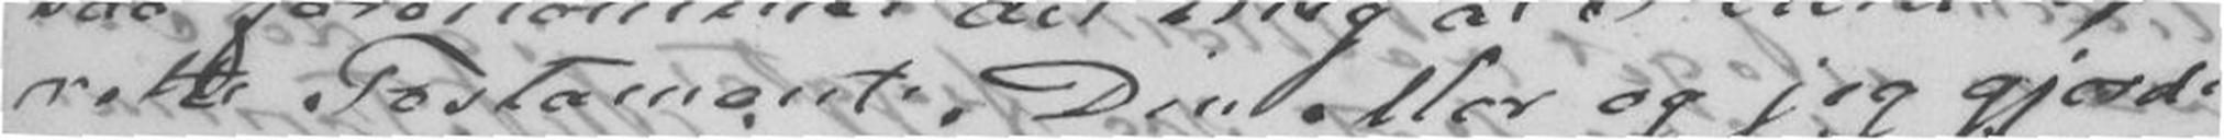rette Testamente, Din Mor og jeg gjorde
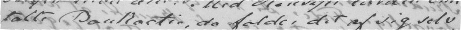talte Bankactier, da falder det af sig selv
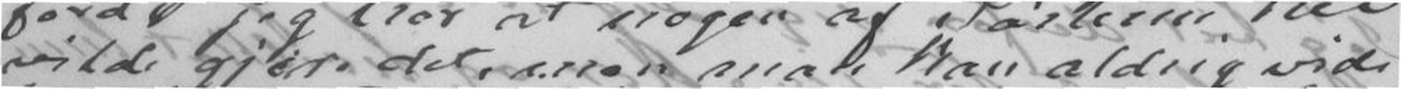vilde gjøre det, men man kan aldrig vide
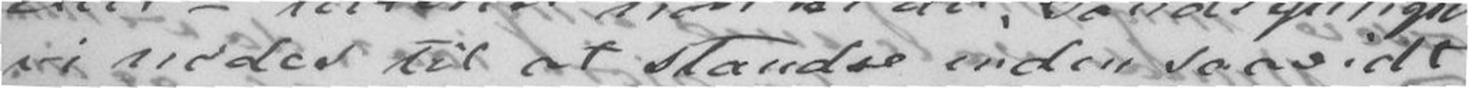vi nødes til at Standse inden saavidt
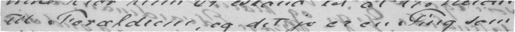til Forældrene, og det jo er en Ting som
